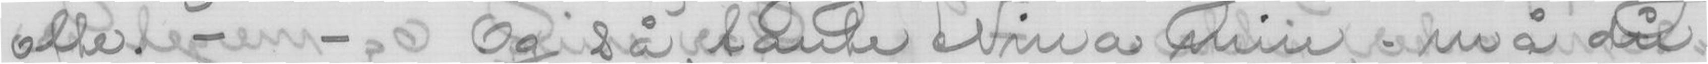ofte. - - Og så tante Nina min - må du
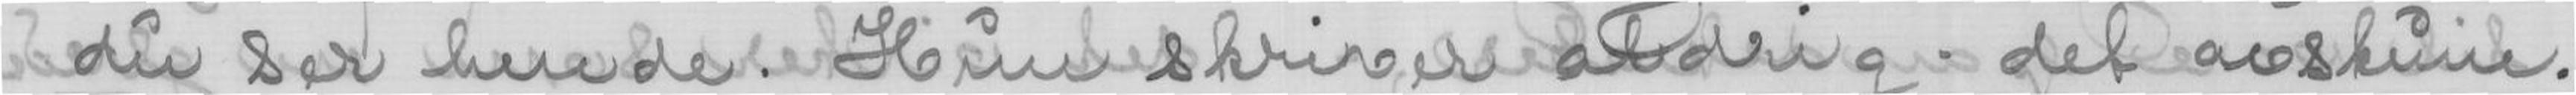du ser hende. Hun skriver aldrig - det avskum.
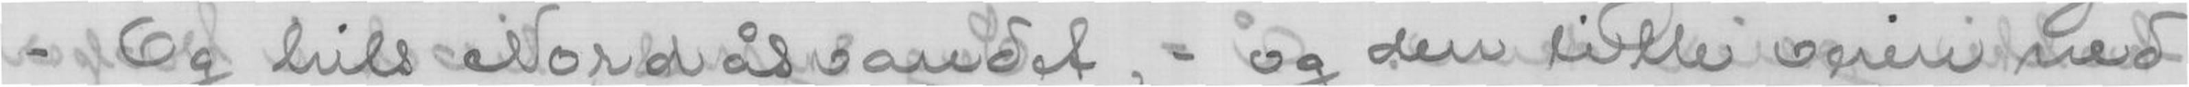- Og hils Nordåsvandet, - og den lille veien ned
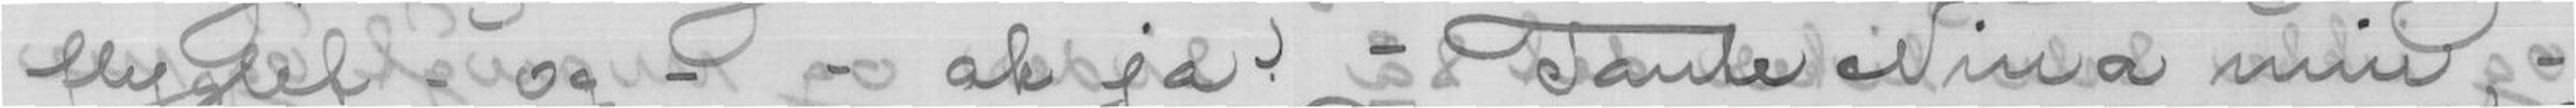flyglet - og - - ak ja! - Tante Nina min, -
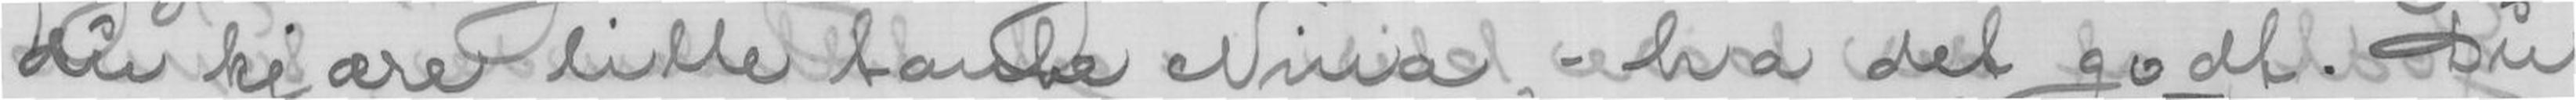du kjære lille tante Nina, - ha det godt. Du
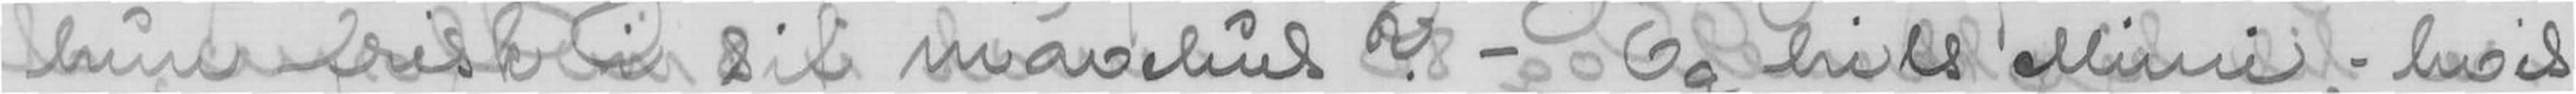hun frisk i sit mavehus? - Og hils Mimi, - hvis
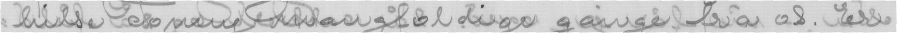hilse Tonny mangfoldige gange fra os. Er
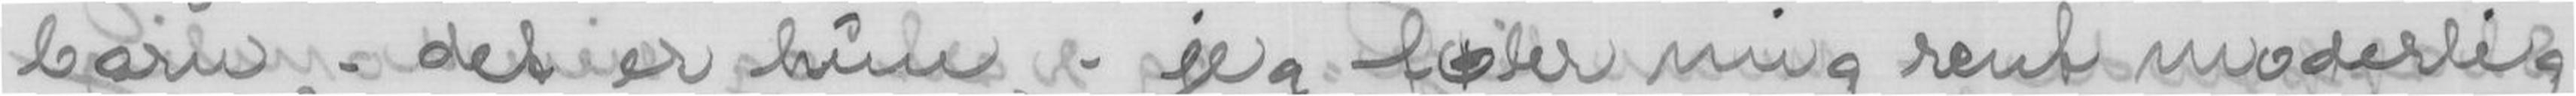barn, - det er hun , - jeg føler mig rent moderlig
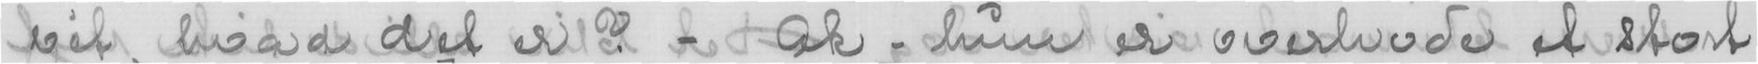vét, hvad det er? - Ak - hun er overhode et stort
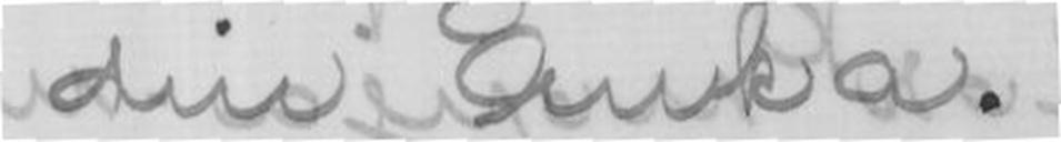din Anka.
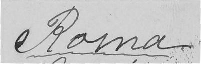Roma
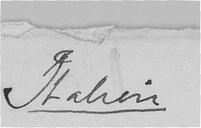Italien
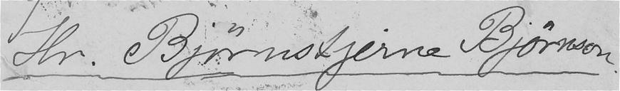Hr. Bjørnstjerne Bjørnson
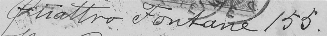Quattro Fontane 155
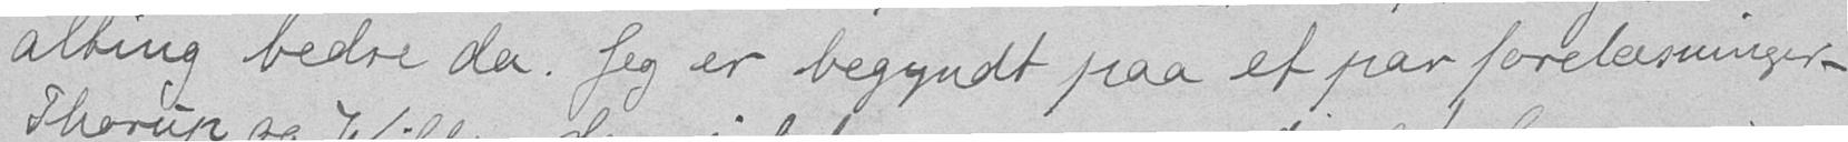allting bedre da. Jeg er begyndt paa et par forelæsninger -
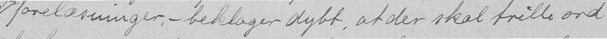forelæsninger, - beklager dybt, at der skal trille ord
